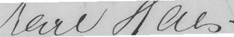Karl Hals.
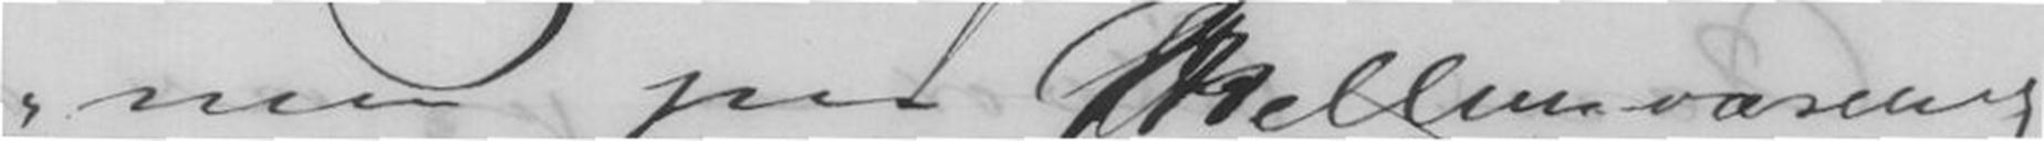men paa Mellemvæsenet
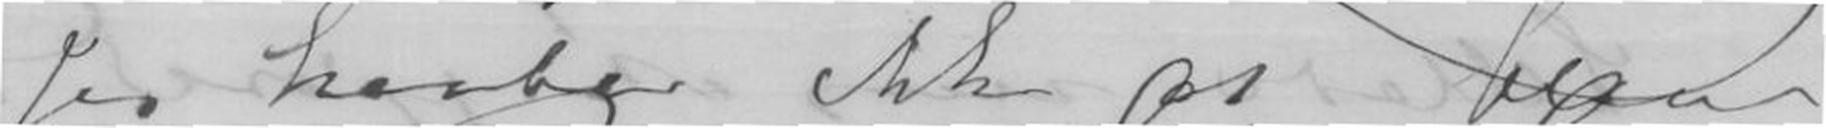Jeg haaber ikke at blive
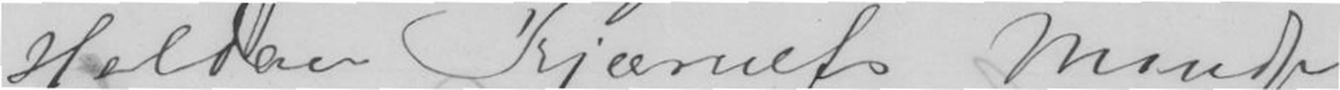Haldan Kjærulfs Minde-
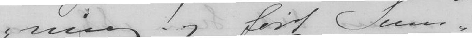ning og ført Sum-
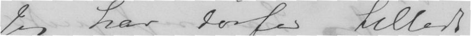Jeg har derfor tilladt
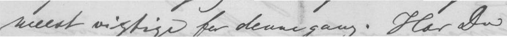mest vigtige for dennegang. Har Du
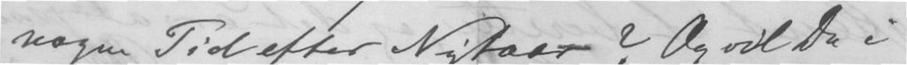nogen Tid efter Nytaar? Og vil Du i
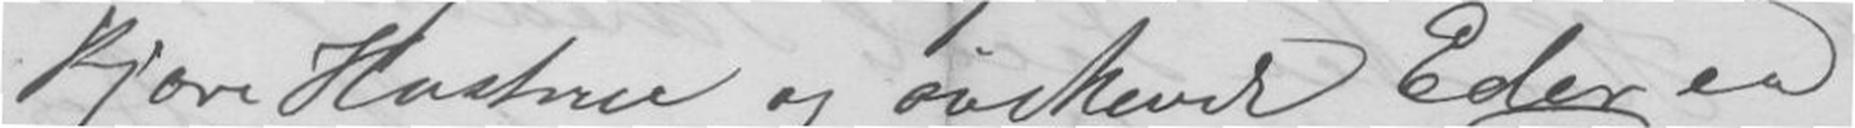kjære Hustru og ønskende Eder en
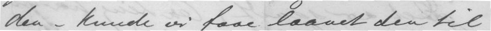den - kunde vi faae laanet den til
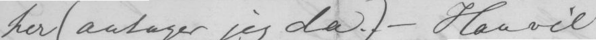her (antager jeg da.) - Han vil
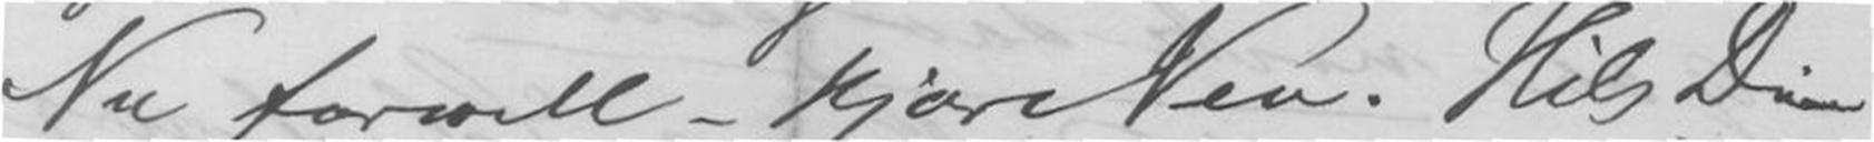Nu farvell - kjære Ven. Hils Din
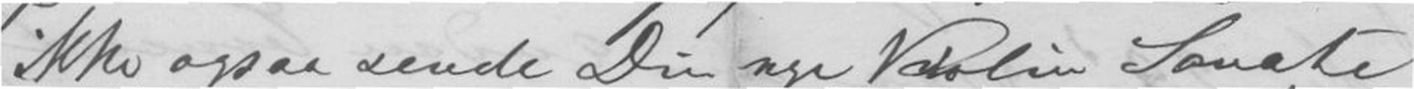ikke ogsaa sende Din nye Violin Sonate
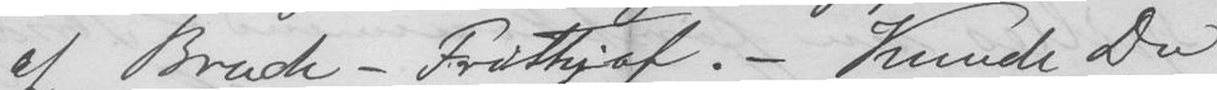af Brade - Frithjof. - Kunde Du
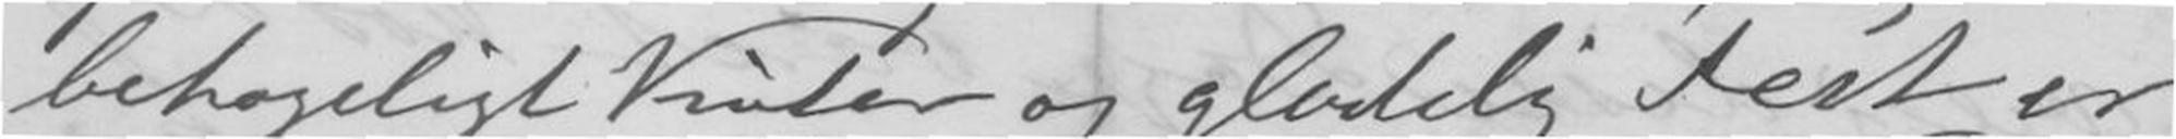behageligt Vinter og glædelig Fest er
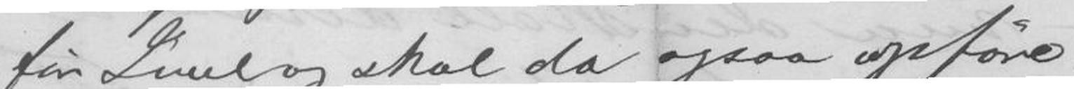før Juul og skal da ogsaa opføre
